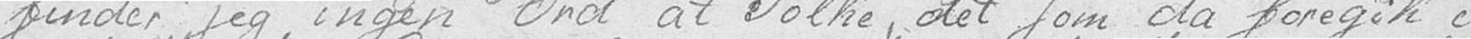finder jeg ingen Ord at Tolke, det som da foregik i
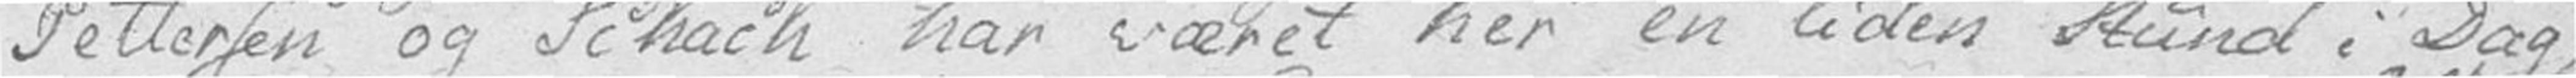Pettersen og Schach har været her en liden Stund i Dag,
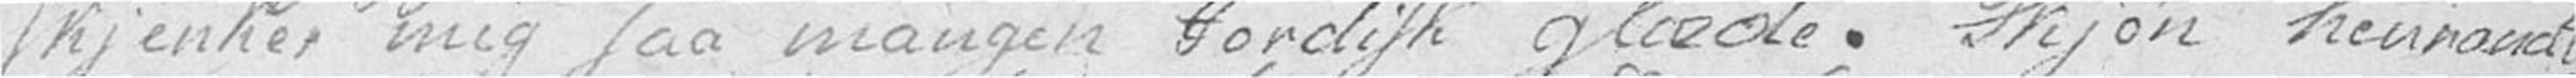skjenker mig saa mangen Jordisk glæde. Skjøn henrandt
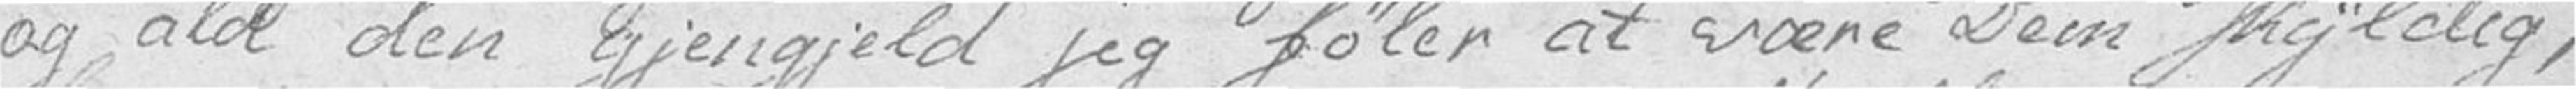og ald den gjengjeld jeg føler at være Dem skyldig,
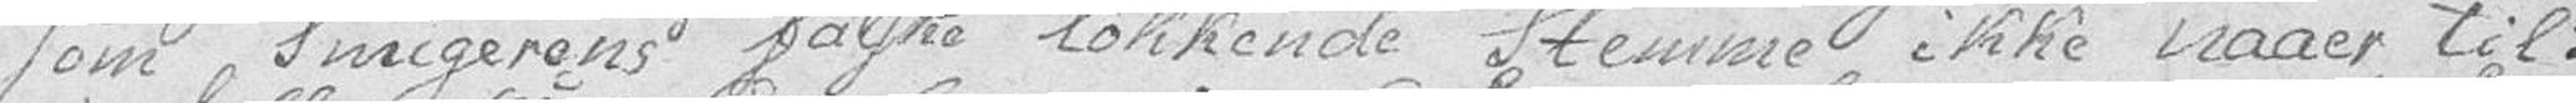som Smigerens falske lokkende Stemme ikke naaer til:
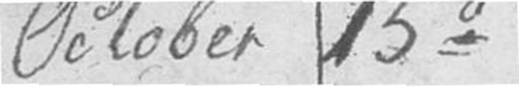October 15d
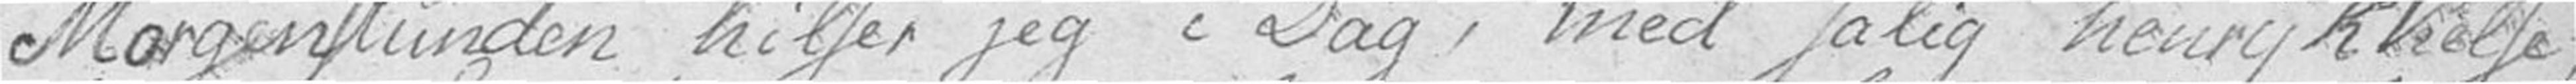Morgenstunden hilser jeg i Dag, med salig henrykkelse,
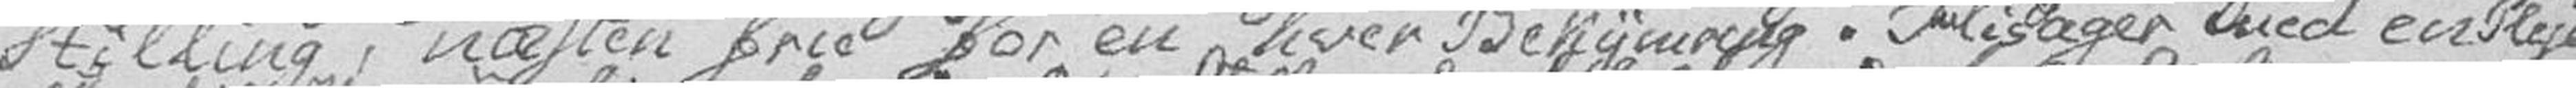Stilling, næsten frie for en hver Bekymring. Flisager med en Pleje
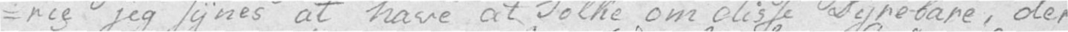-rie jeg synes at have at Tolke om disse Dyrebare, der
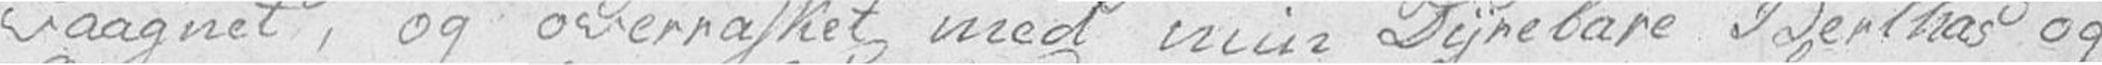vaagnet, og overrasket med min Dyrebare Berthas og
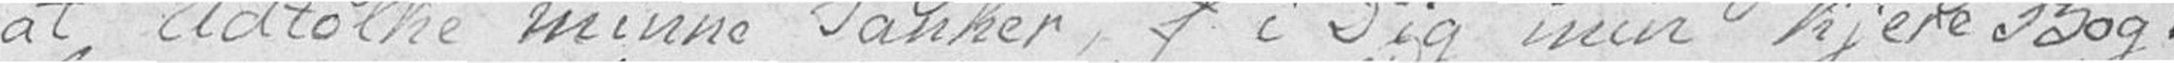at udtolke minne Tanker, f i Dig min kjere Bog:
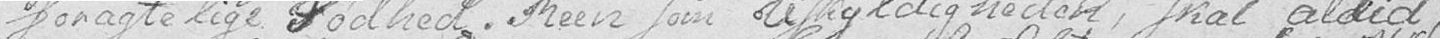foragtelige Sødhed. Reen som Uskyldigheden, skal altid
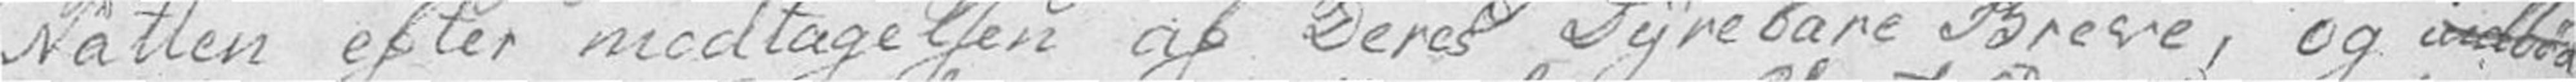Natten efter modtagelsen af Deres Dyrebare Breve, og indbød
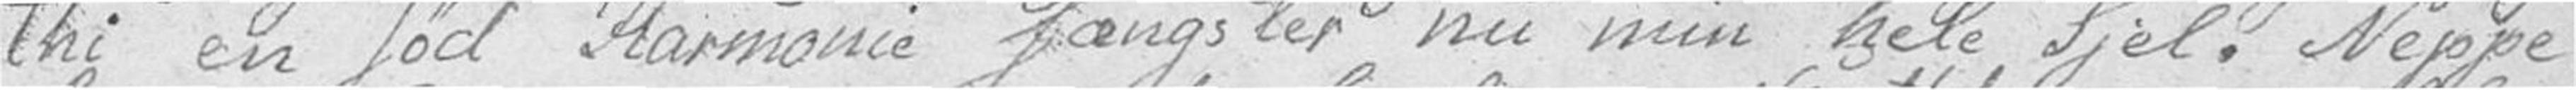thi en sød Harmonie fængsler nu min hele Sjel. Neppe
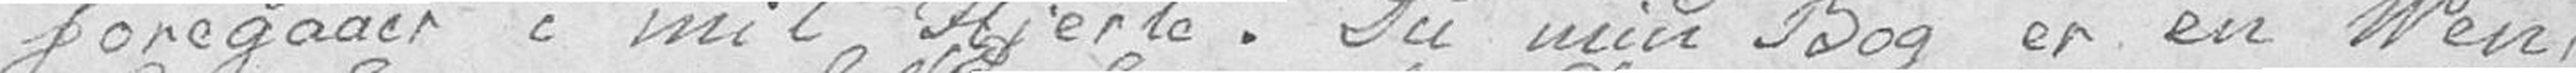foregaaer i mit Hjerte. Du min Bog er en Ven,
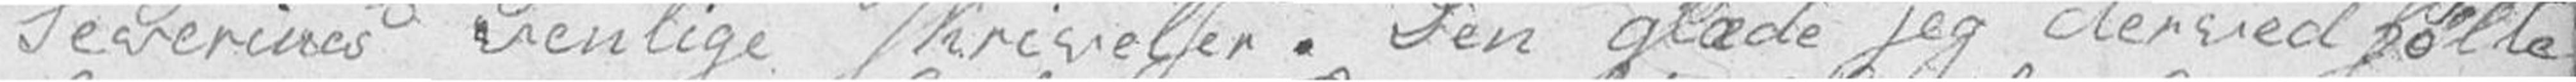Severines venlige skrivelser. Den glæde jeg derved følte,
